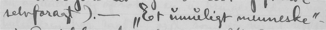selvforagt), - "Et umuligt menneske" -
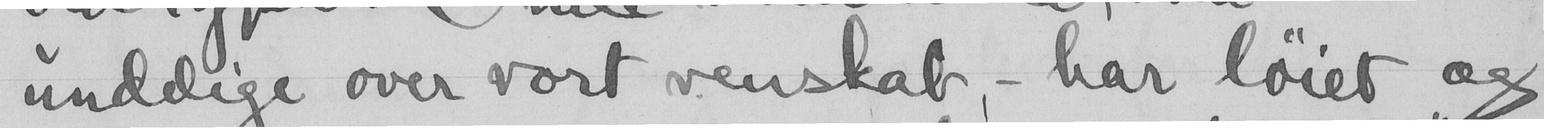unddige over vort venskab - har løiet og
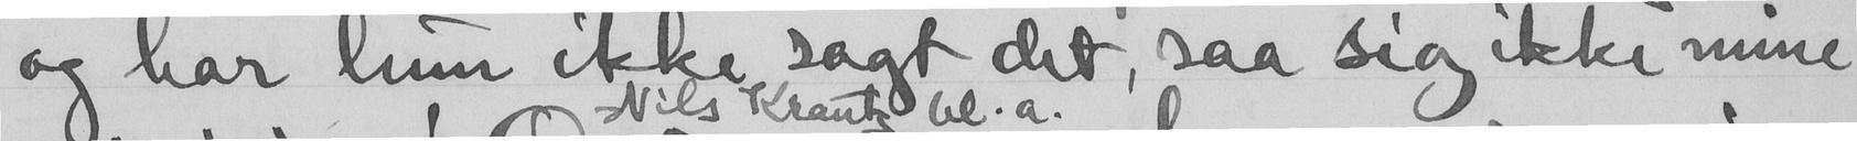og har hun ikke saat det, saa sig ikke mine
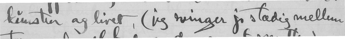kunsten og livet (jeg svinger jo stadig mellem
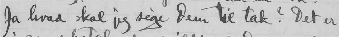Ja hvad skal jeg sige Dem til tak? Det er
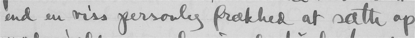end en viss personlig frækhed at satte op
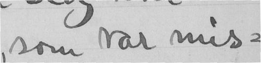som var mis-
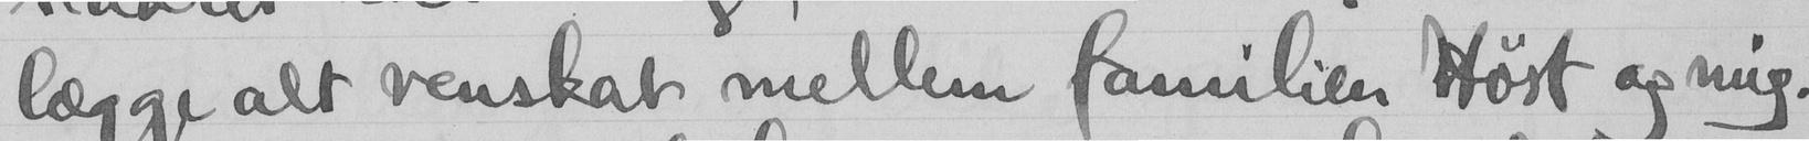lægge alt venskab mellem familien Høst og mig.
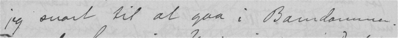jeg snart til at gaa i Barndommen.
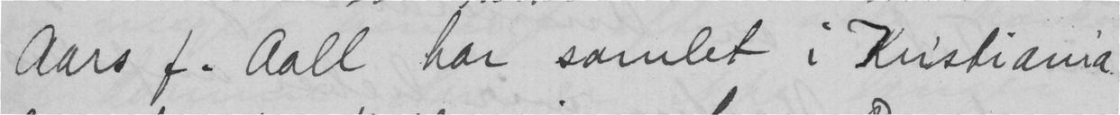Aars f. Aall har samlet i Kristiania.
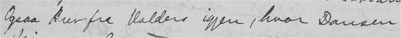Ogsaa Brev fra Valders igjen, hvor Dansen
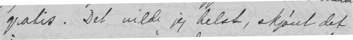gratis. Det vilde jeg helst, skjønt det
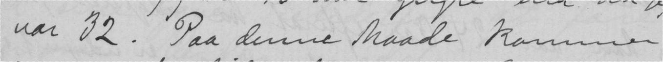var 32. Paa denne Maade kommer
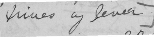trives og lever.
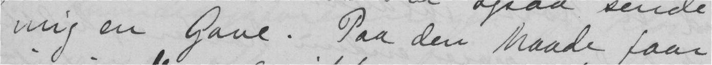mig en Gave. Paa den Maade faar
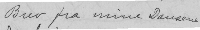Brev fra mine Dansene
